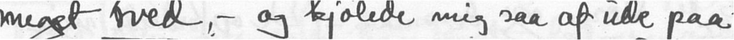meget sved, - og kjølede mig saa af ude paa
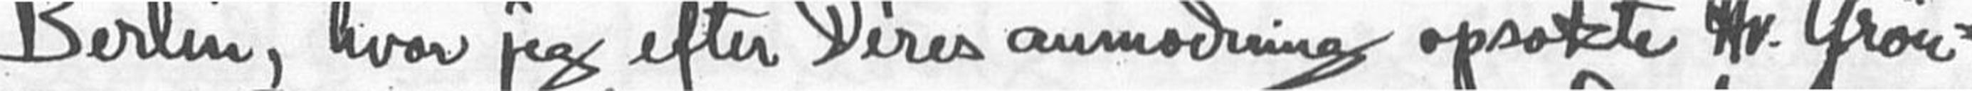Berlin, hvor jeg efter Deres anmodning opsøkte Hr. Grøn-
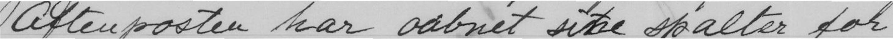Aftenposten har aabnet sine spalter for
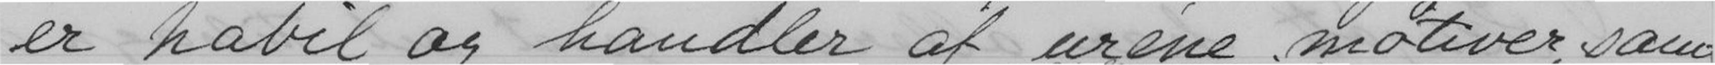er habil og handler af urene motiver, samt
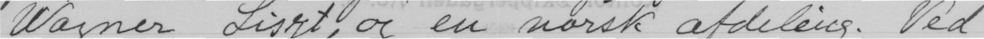Wagner Liszt, og en norsk afdeling. Ved
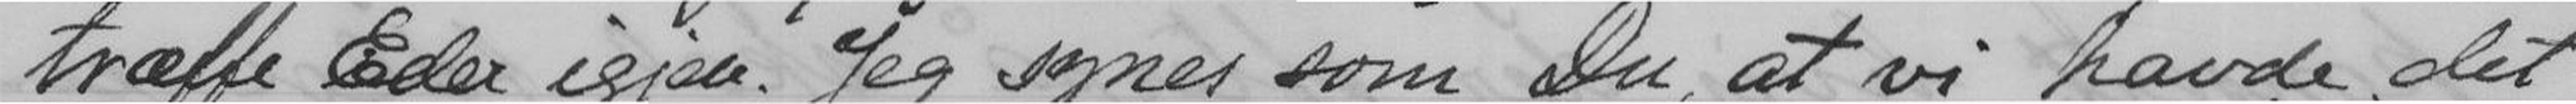træffe Eder igjen. Jeg synes som Du, at vi havde det
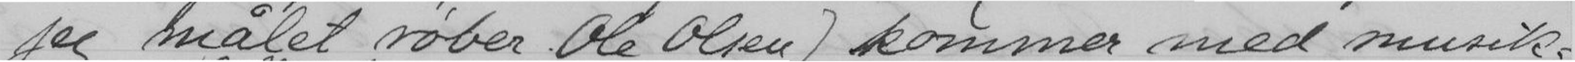jeg målet røber Ole Olsen) kommer med musik-
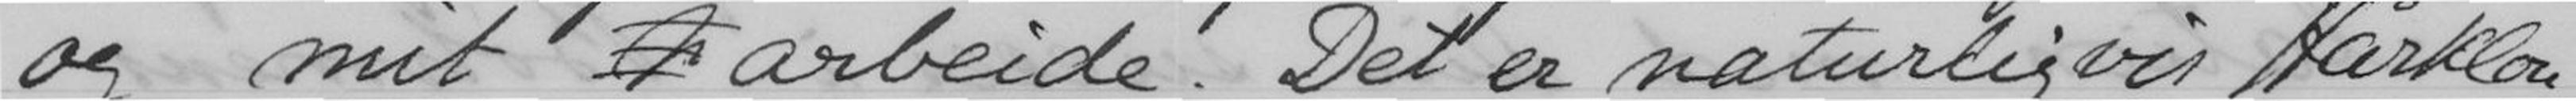og mit arbeide. Det er naturligvis Hårklou
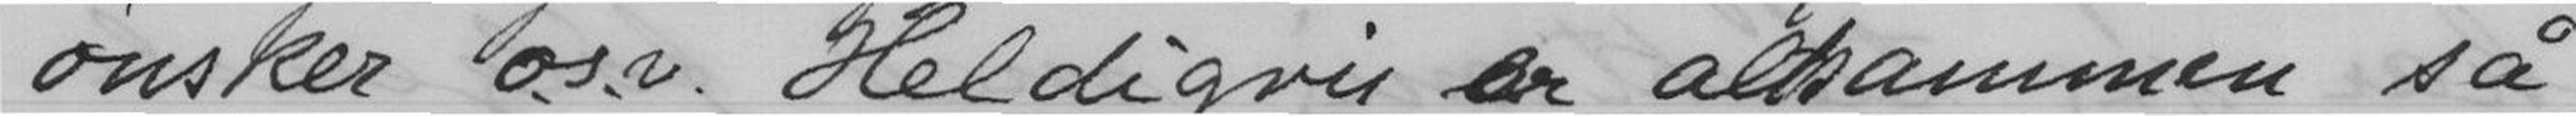ønsker o.s.v. Heldigvis er altsammen så
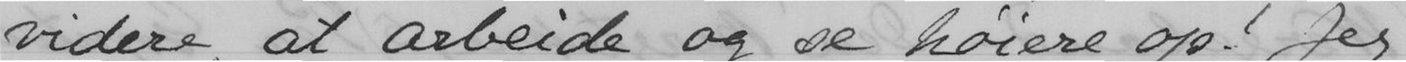videre at arbeide og se høiere op! Jeg
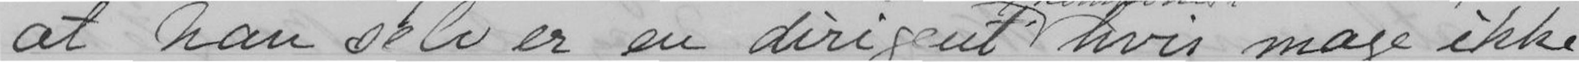at han selv er en dirigent hvis mage ikke
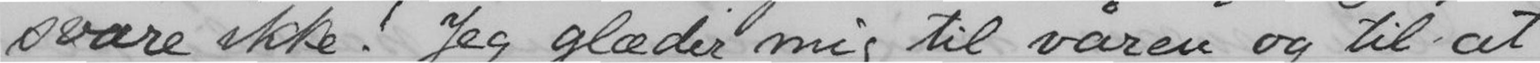svare ikke! Jeg glæder mig til våren og til at
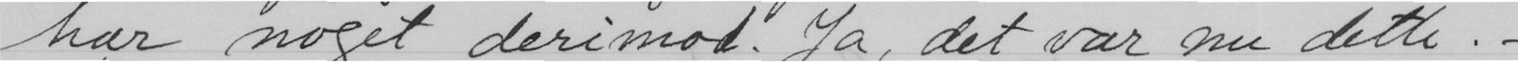har noget derimod. Ja, det var nu dette. -
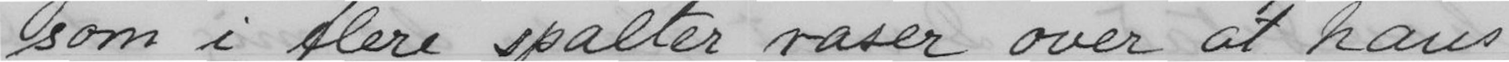som i flere spalter raser over at hans
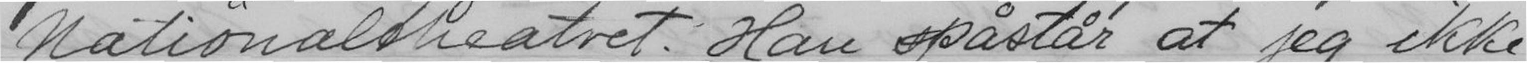Nationaltheatret. Han påstår at jeg ikke
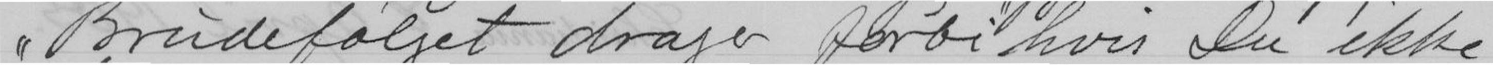Brudefølget drager forbi hvis Du ikke
